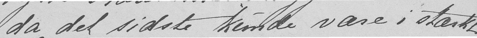da det sidste kunde være i stærkt
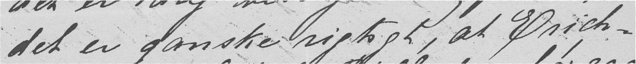det er ganske rigtigt, at Erich-
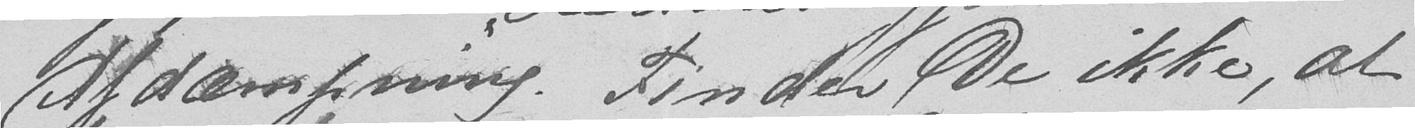Afdæmpning. Finder De ikke, at
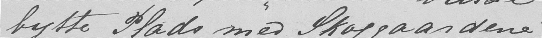bytte Plads med "Skoggaardene"
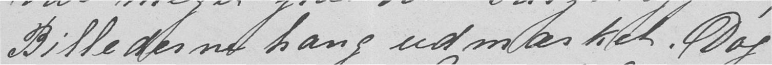Billederne hang udmærket. Dog
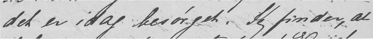det er idag besørget. Jeg finder, at
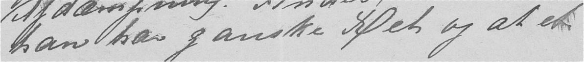han har ganske Ret og at et
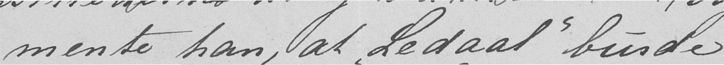mente han, at "Ledaal" burde
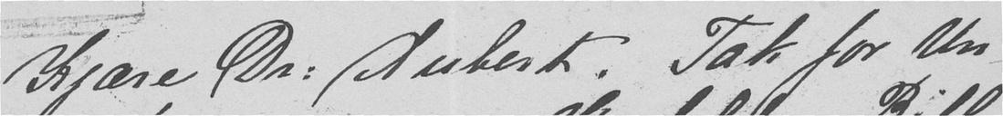Kjære Dr Aubert. Tak for Un-
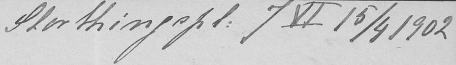Storthingspl. 7 VI 15/4 1902
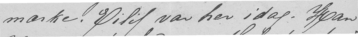mærke. Eilif var her idag. Han
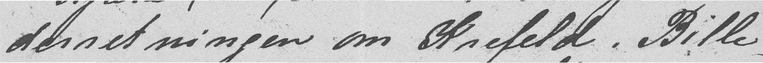derretningen om Krefeld. Bille-
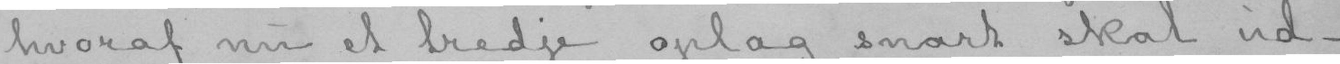hvoraf nu et tredje oplag snart skal ud-
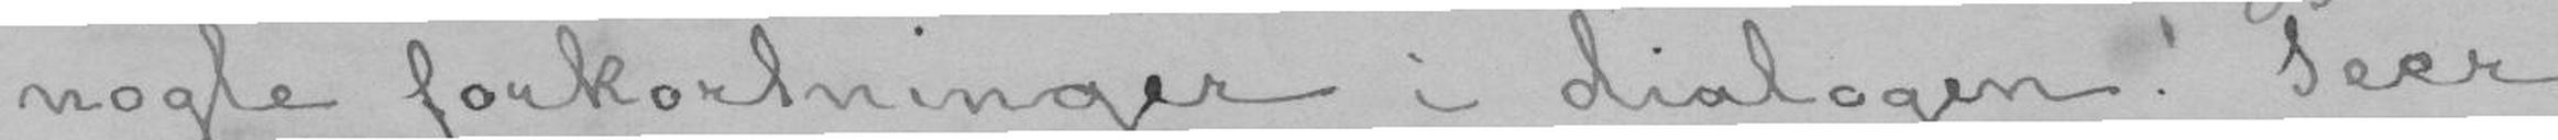nogle forkortninger i dialogen. Peer
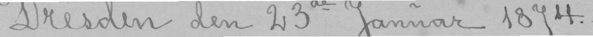Dresden den 23de Januar 1874.
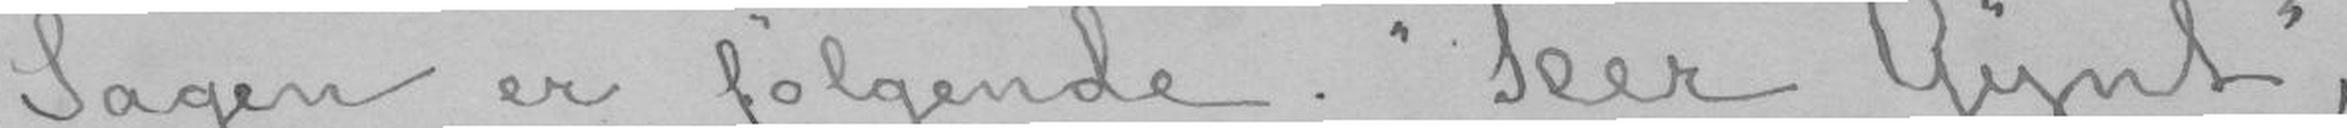Sagen er følgende "Peer Gynt",
